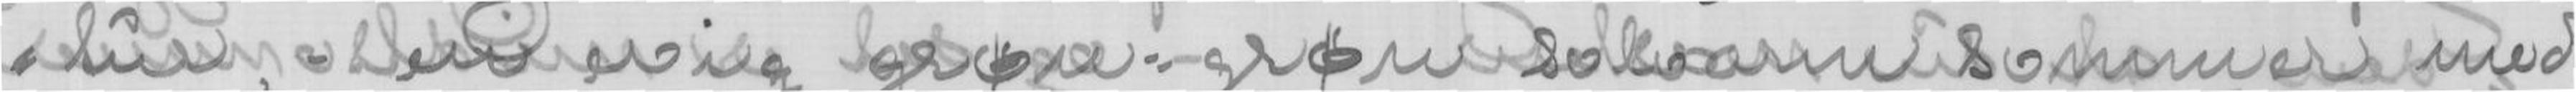tur, - en evig grøn-grøn solvarm sommer med
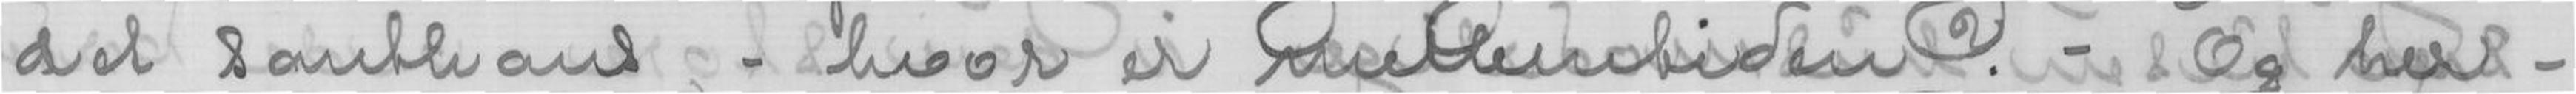det santhans, - hvor er mellemtiden? - Og her -
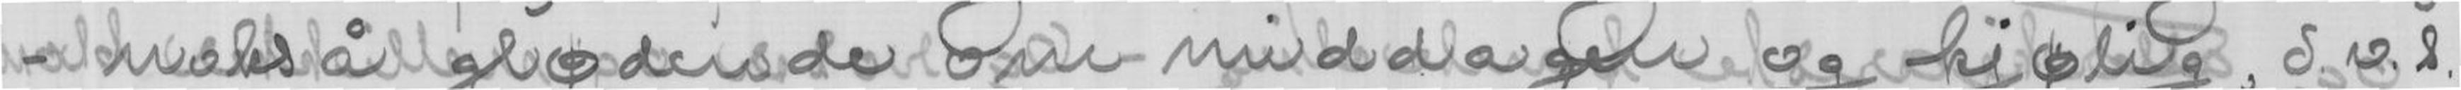- nokså glødende om middagen og kjølig, d.v.s.
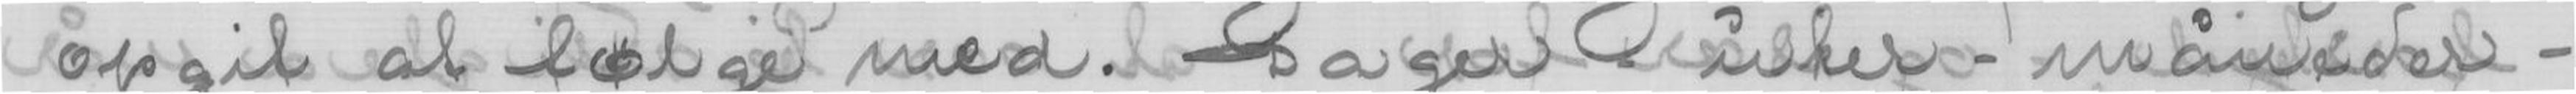opgit at følge med. Dager - uker - måneder -
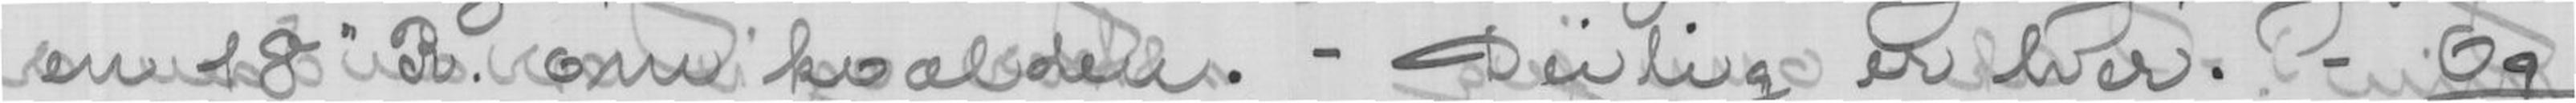en 18" R. om kvælden. - Deilig er her. - Og
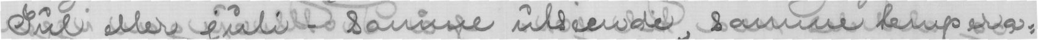Jul eller juli - samme utseende, samme tempera-
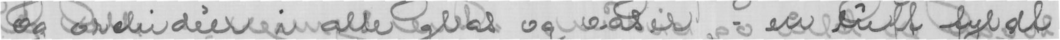og orchidéer i alle glas og vaser, - en luft fyldt
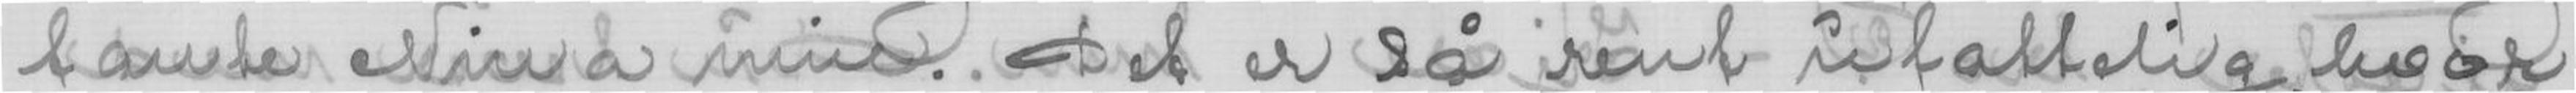tante Nina min.. Det er så rent ufattelig, hvor
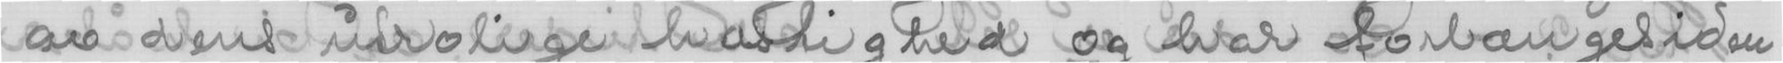av dens utrolige hastighed og har forlængesiden
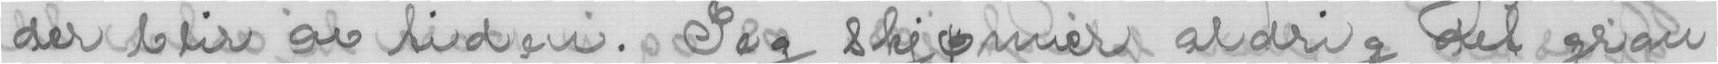der blir av tiden. Jeg skjønner aldrig det gran
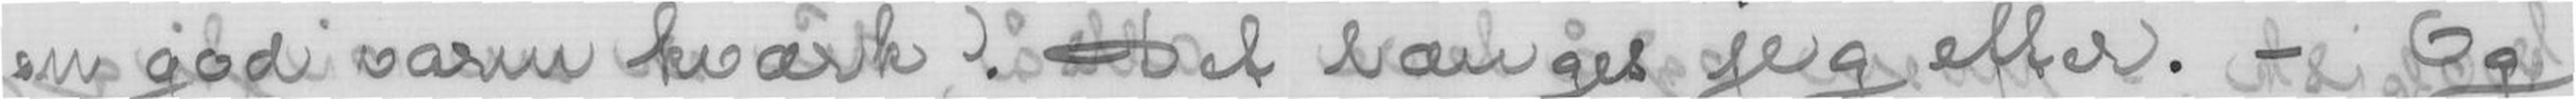en god varm kværk! Det længes jeg efter. - Og
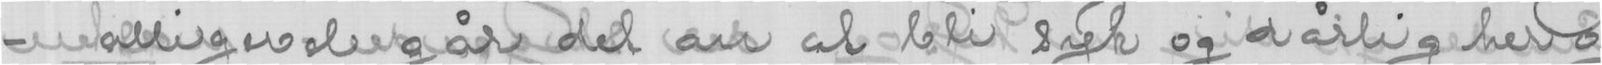- alligevel går det an at bli syk og dårlig her og.
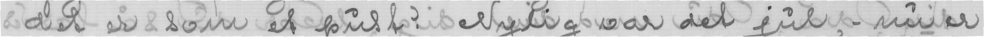det er som et pust! Nylig var det jul, nu er
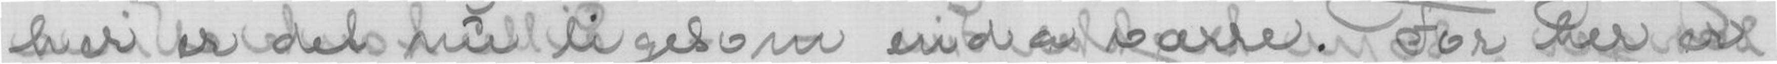her er det nu ligesom enda værre. For her er
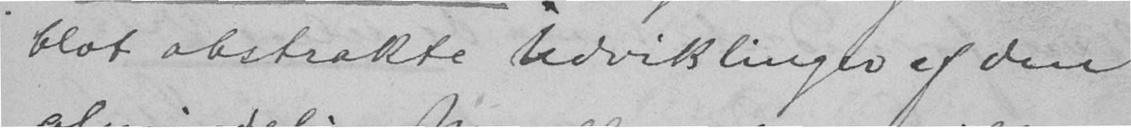blot abstrakte Udviklinger af den
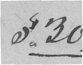§ 30.
